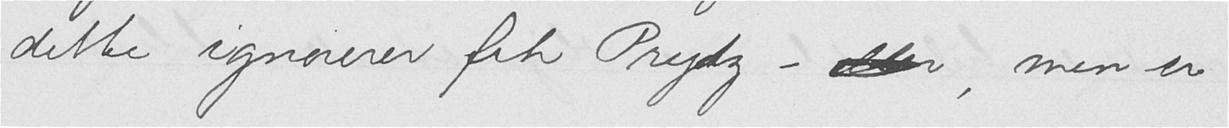dette ignorerer frk Prydz - der, men er
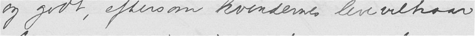og godt, eftersom kvindernes levevilkaar
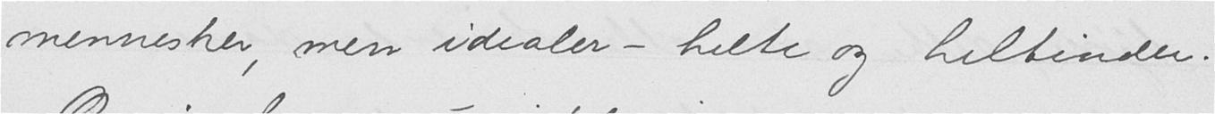mennesker, men idealer - helte og heltinder.
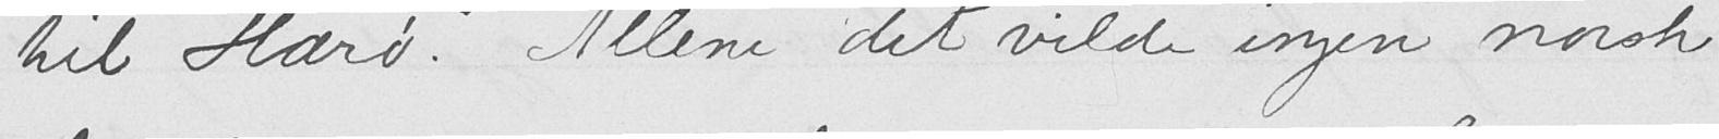til Hærø". Allene det vilde ingen norsk
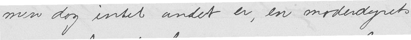men dog intet andet er, en moderdyrets
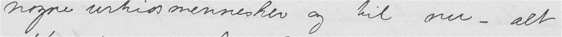nøgne urtidsmennesker og til nu - alt
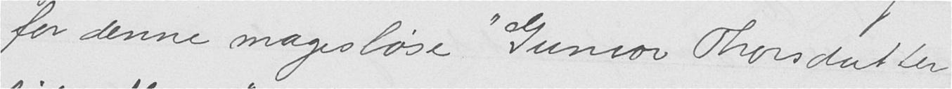for denne magesløse "Gunvor Thorsdatter
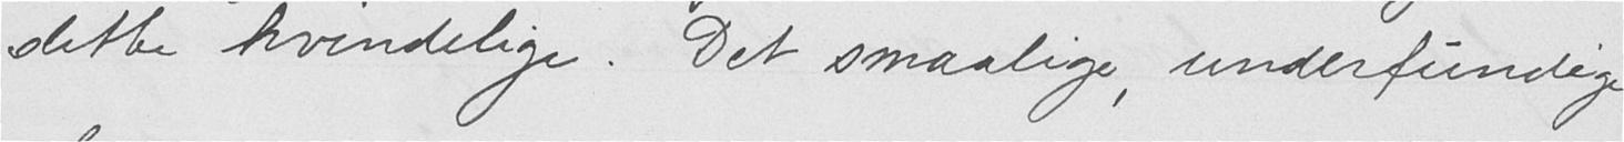dette kvindelige. Det smaalige, underfundige
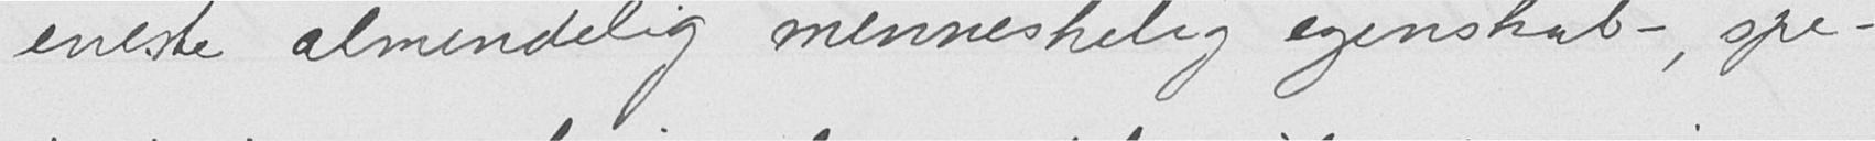eneste almindelig menneskelig egenskab -, spe-
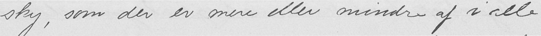sky, som der er mere eller mindre af i alle
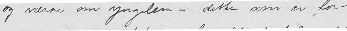og værne om yngelen - dette som er for-
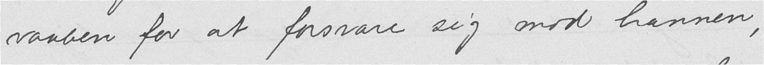vaaben for at forsvare sig mod hannen,
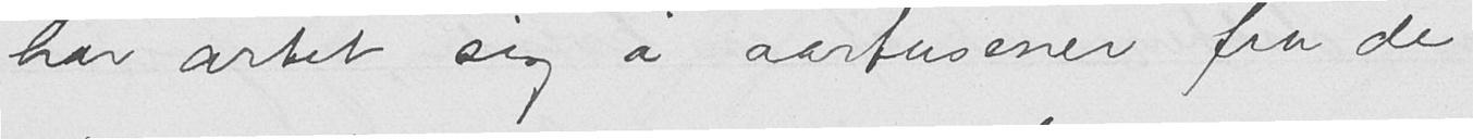har artet sig i aartusener fra de
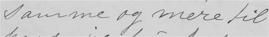samme og mere til
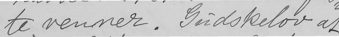te venner. Gudskelov at
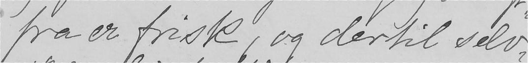fra er frisk, og dertil selv-
